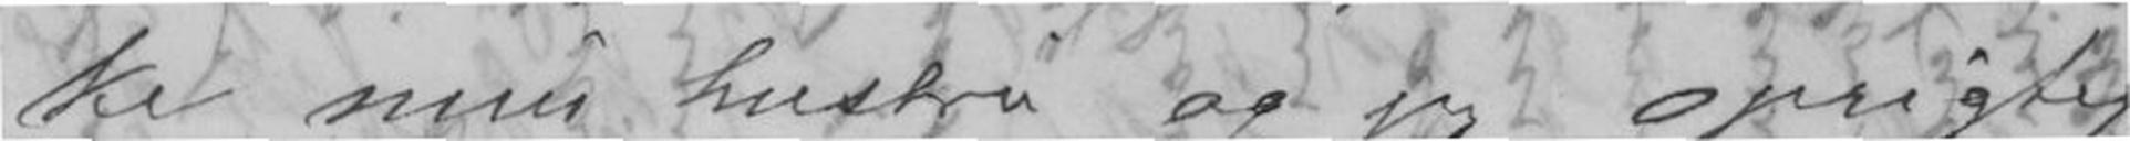ke min hustru og jeg oprigtig
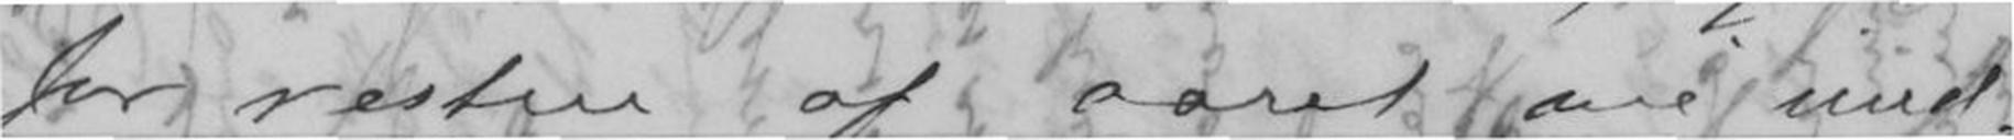for resten af aaret vil und-
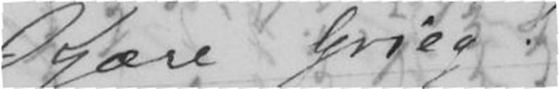Kjære Grieg!
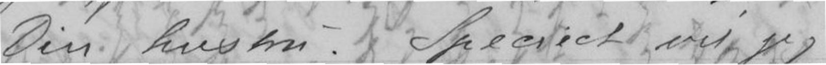Din hustru. Specielt vil jeg
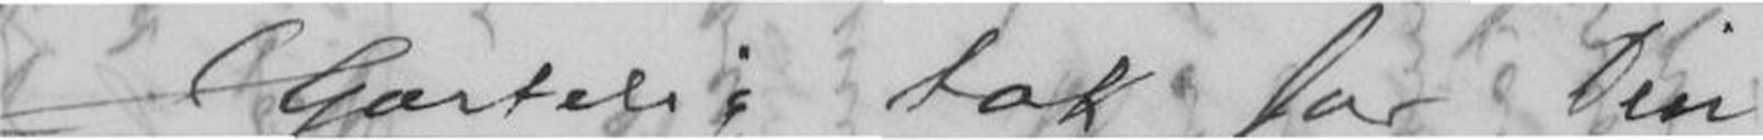Hjærtelig tak for Din
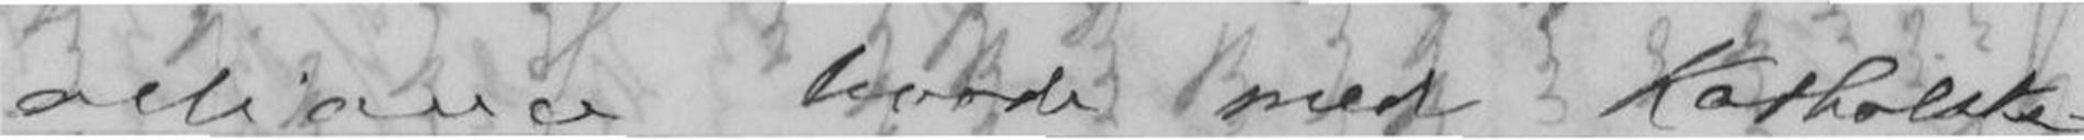seliance lovede mest katolske
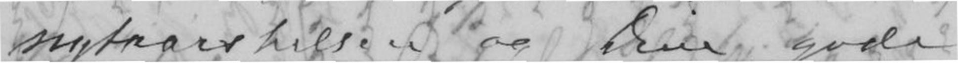nytaarshilsen og Dine gode
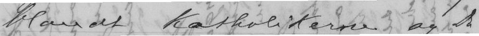blandt katholikerne, og Du
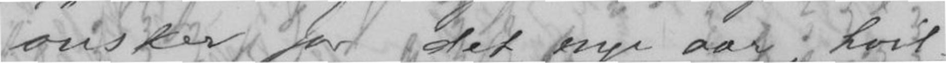ønsker for det nye aar; hvil-
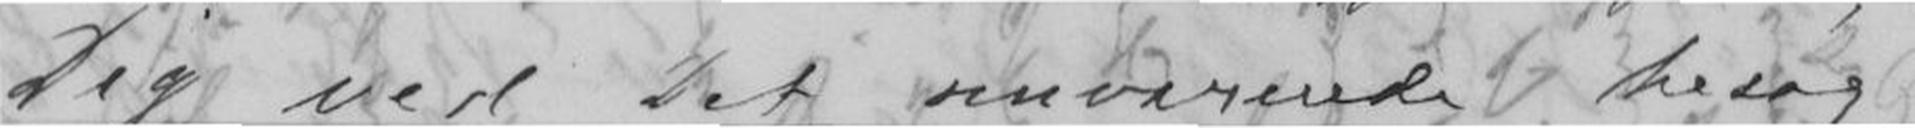Dig ved Det nuværende besøg
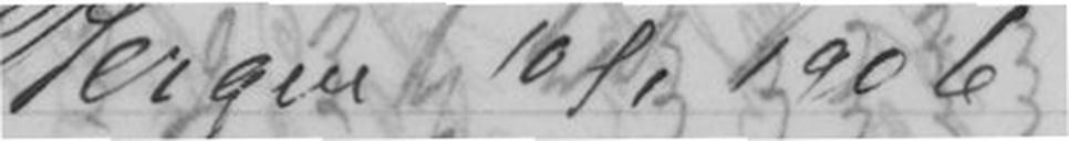Bergen 10/6 1906
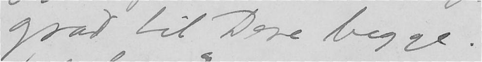grad til Dere begge.
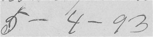5 - 4 - 93
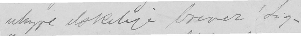uhyre elskelige brever. Lig-
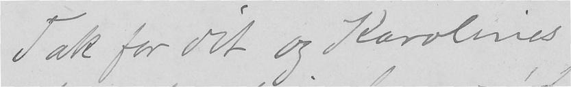Tak for dit og Karolines
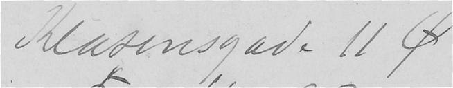Klasensgade 11 Ø
